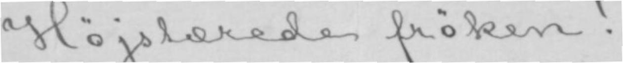Højstærede frøken!
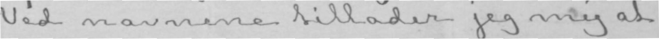Ved navnene tillader jeg mig at
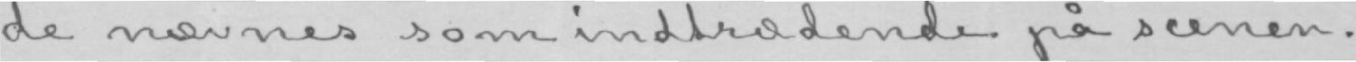de nævnes som indtrædende på scenen.
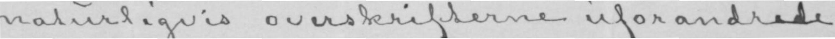naturligvis overskrifterne uforandrede,
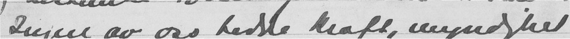Ingen av oss hadde kraft, myndighet
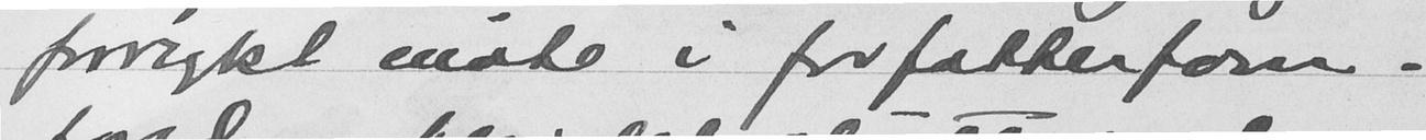forrykt møte i forfatterforn.
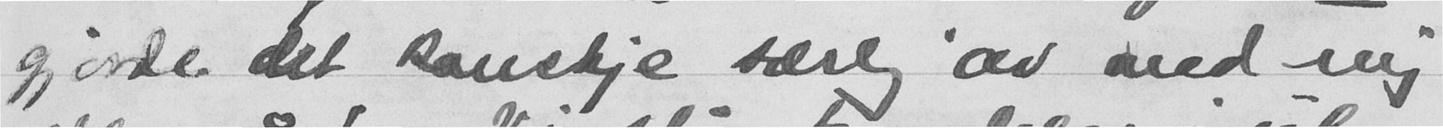gjorde det kanskje særlig av ned mig
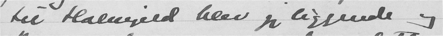til Holmegild blev jeg liggende og
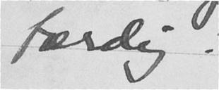ferdig!
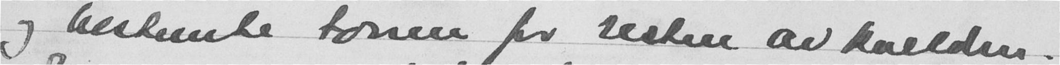og bestemte tonen for resten av kvelden.
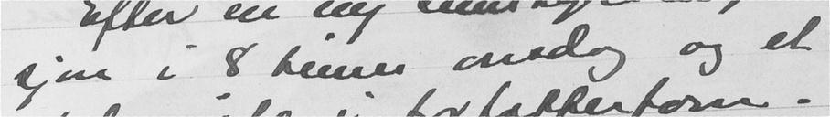sjon i 8 timer onsdag og et
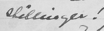stillinger!
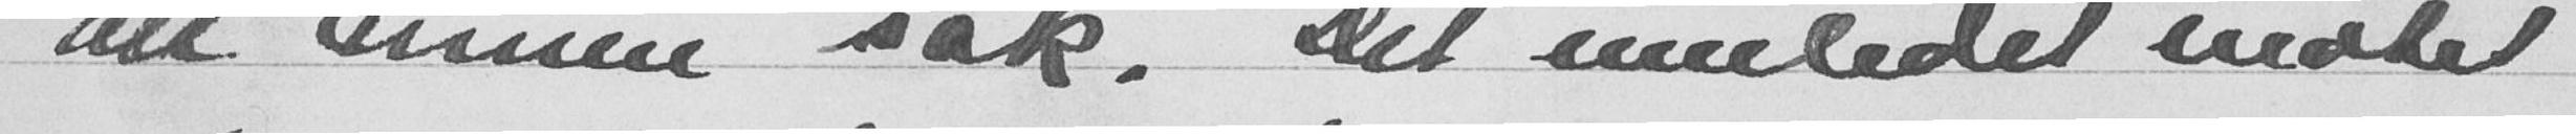all annen sak. Det innledet møtet
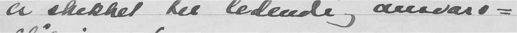er skikket til ledende og ansvars-
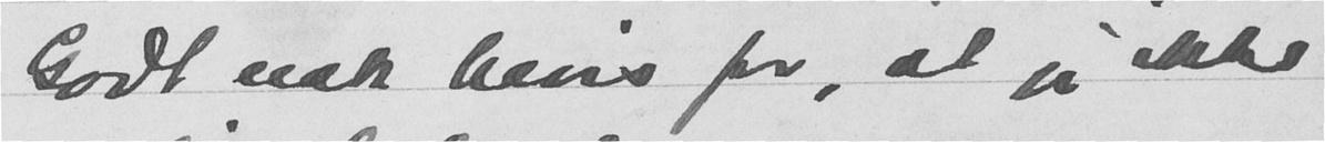Godt nok bevis for, at jeg ikke
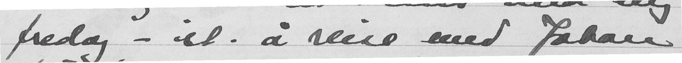fredag - et. å reise med Johan
